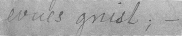evnes gnist;-
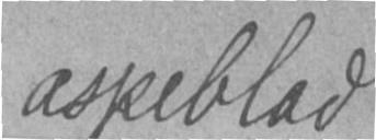aspeblad
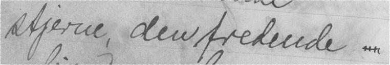stjerne, den fredende -
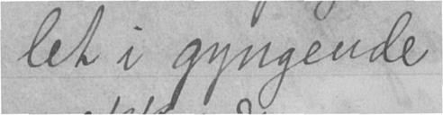let i gyngende
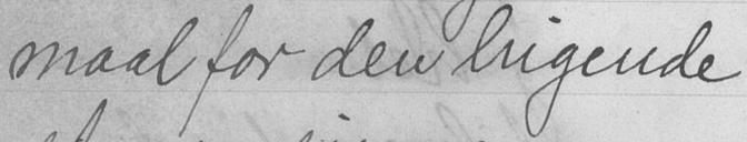maal for den higende
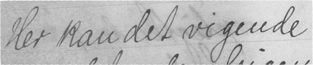Her kan det vigende
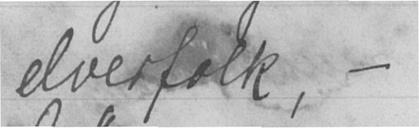elverfolk, -
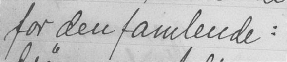for den famlende:
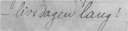- livsdagen lang!
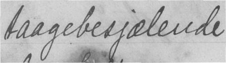taagebesjælende
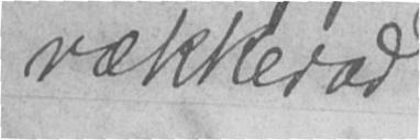rækkerad
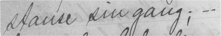stause sin gang; -
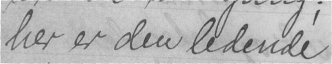her er den ledende
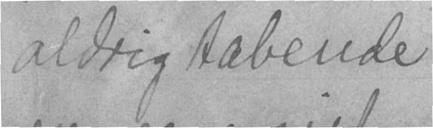aldrig tabende
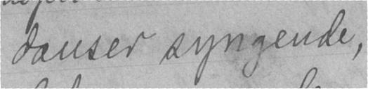danser syngende,
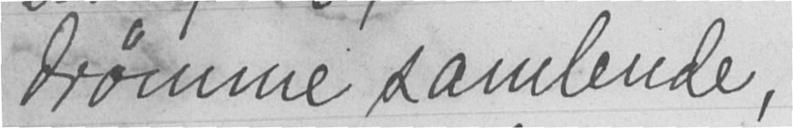drømme samlende,
